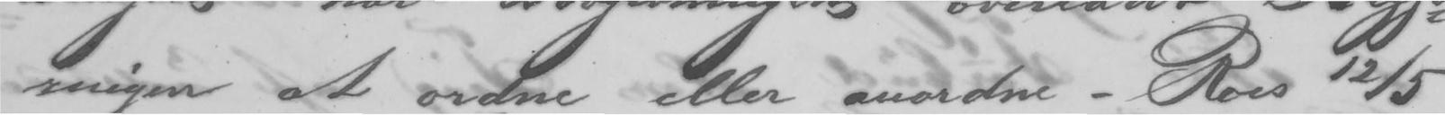ringen at ordne eller anmodne. Res 12/5
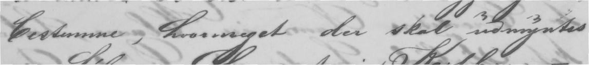bestemme, hvormeget der skal udmyntes
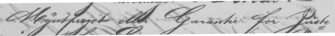Myntpræget eller Garantie for Juste-
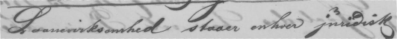Laanevirksomhed staaer enhver juridisk
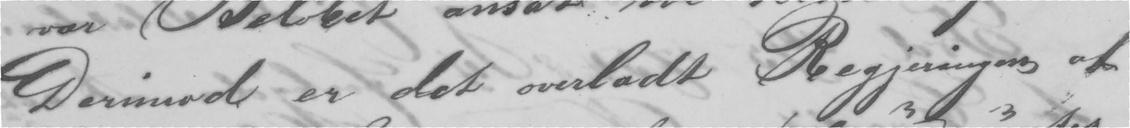Derimod er det overladt Regningen at
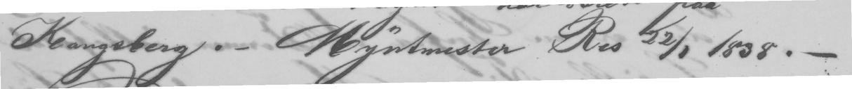Kongsberg. - Myntmester Res 22/1 1838. -
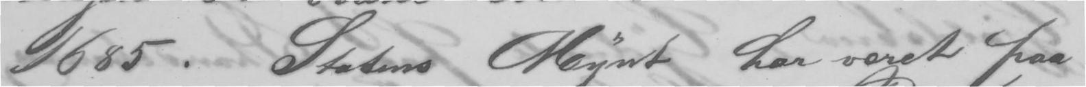1685. Statens Mynt har været paa
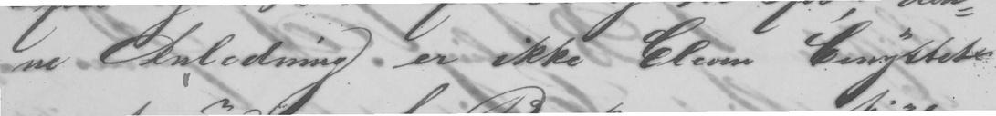ne Inledning en ikke bleven benyttet
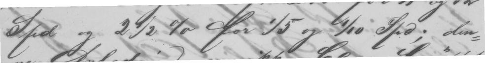Spd og 2½ % for ¹⁄₅ og ¹⁄₁₀ Spd; den-
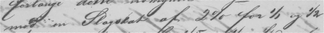mod en Slogskat af 2% for ¹⁄₁ og ½
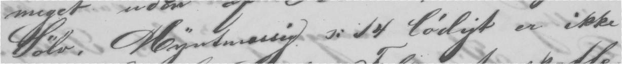Sølv. Myntmessig ɔː14 løligt en ikke
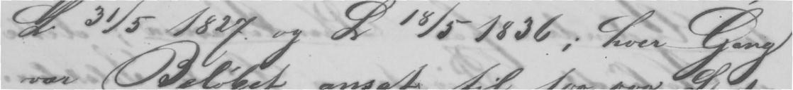L. 31/5 - 1827 og L 18/5 1836; hver Gang
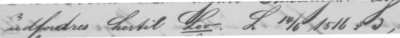udfordres hertil Lov. L. 14/6 1816  §3,
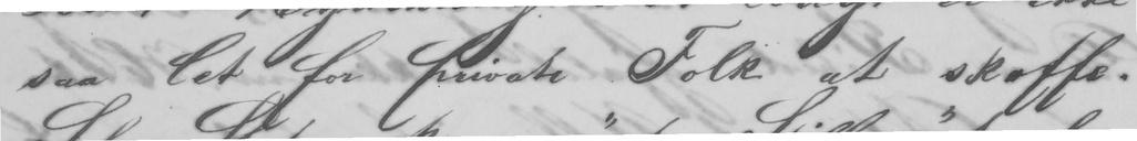saa let for private Folk at skaffe.
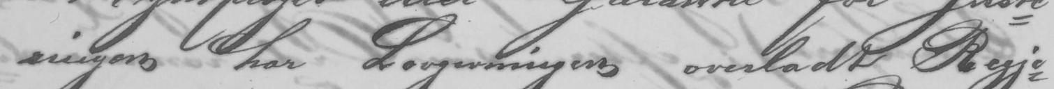ringen har lovgivningen overladt Regje-
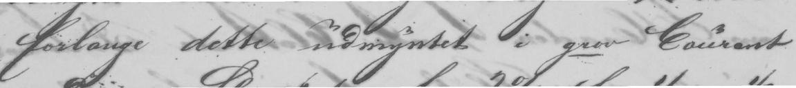forlange dette udmyntet i grov Courant
